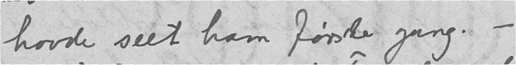havde seet ham første gang. -
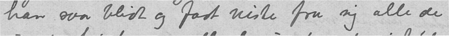han saa blidt og fast viste fra sig alle de
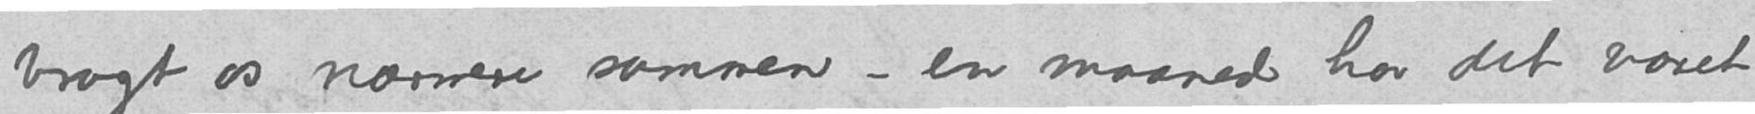bragt os nærmere sammen - en maaned har det været
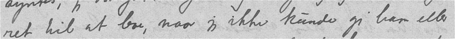ret til at leve, naar jeg ikke kunde gi ham eller
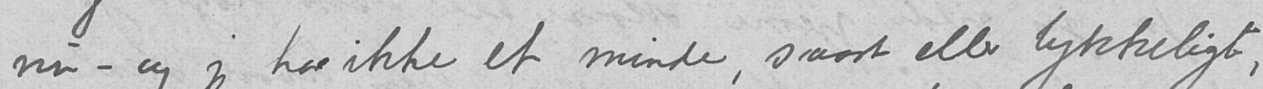nu - og jeg har ikke et minde, saart eller lykkeligt,
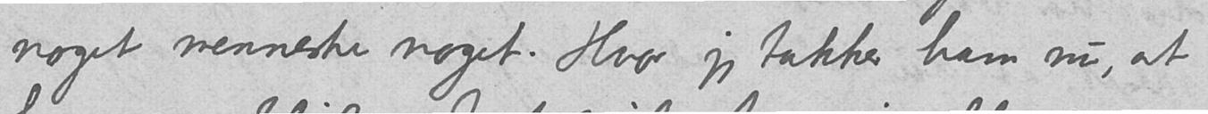noget menneske noget. Hvor jeg takker ham nu, at
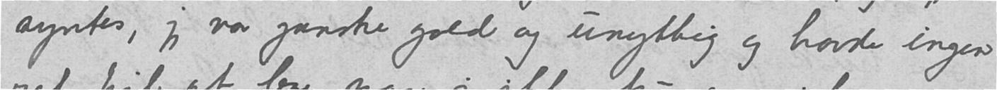syntes, jeg var ganske gold og unyttig og havde ingen
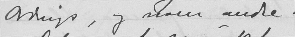Ordings, og noen andre.
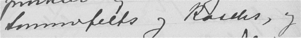Sommerfelts og Raschs, og
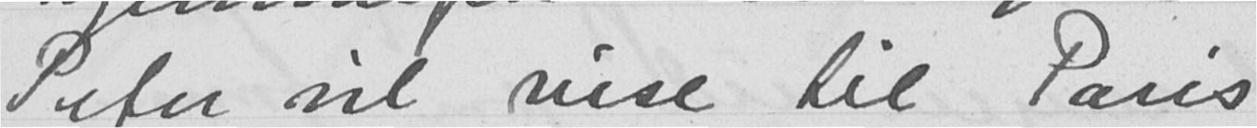Peter vil reise til Paris
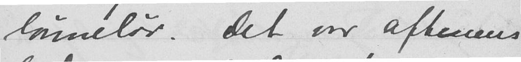lønneløv. Det var aftenens
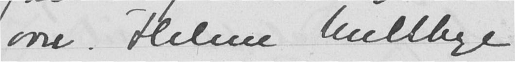ovn. Helene Mellbye
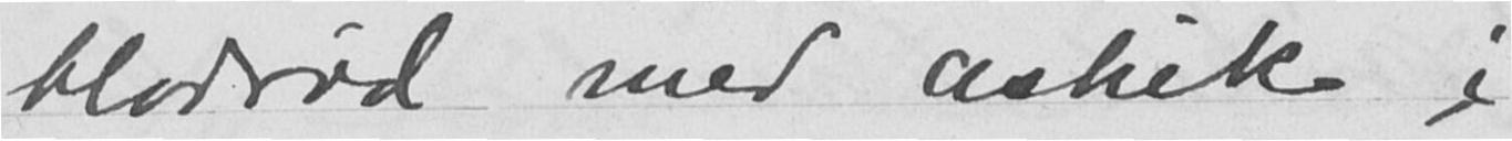blodrød med Astrik i
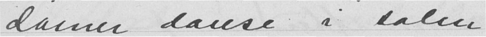damer danse i salen
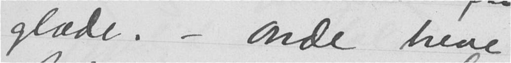glæde. - Onde breve
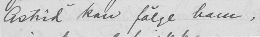Astrid kan følge ham,
